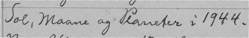Sol, Maane og Planeter i 1944.
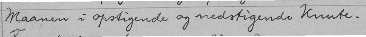Maanen i opstigende og nedstigende Knute.
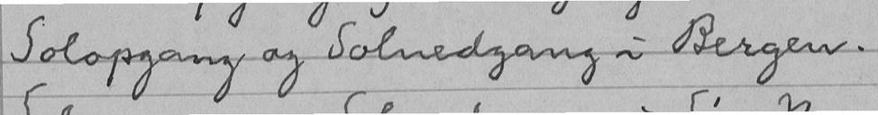Solopgang og Solnedgang i Bergen.
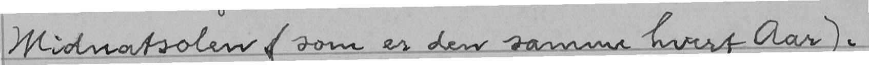Midnatsolen (som er den samme hvert Aar).
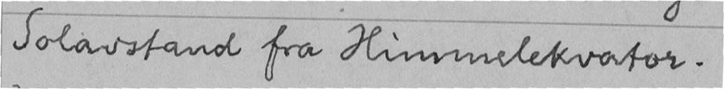Solavstand fra Himmelekvator.
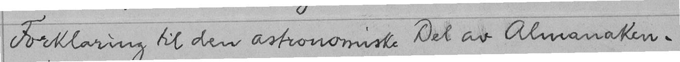Forklaring til den astronomiske Del av Almanaken.
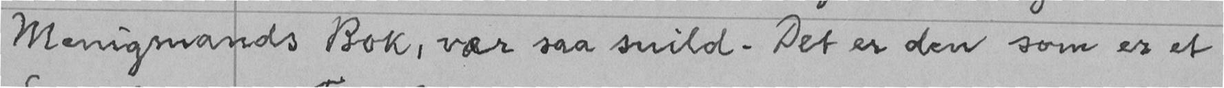Menigmands Bok, vær saa snild. Det er den som er et
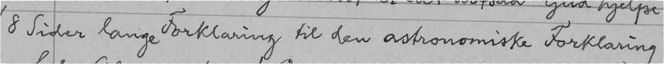8 Sider lange Forklaring til den astronomiske Forklaring
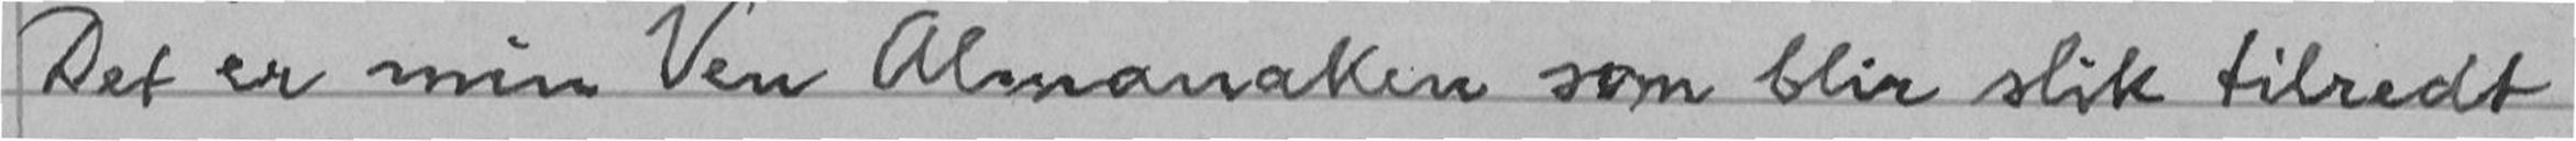Det er min Ven Almanaken som blir slik tilredt
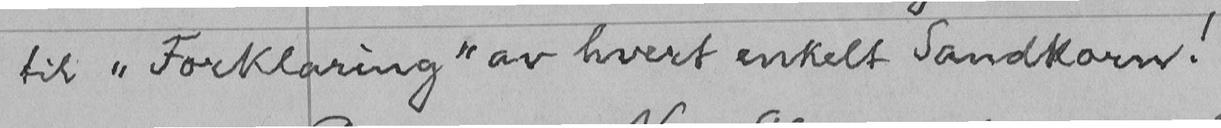til "Forklaring" av hvert enkelt Sandkorn!
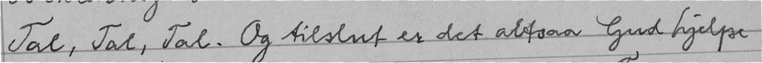Tal, Tal, Tal. Og tilslut er det altsaa Gud hjelpe
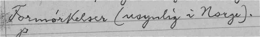Formørkelser (usynlig i Norge).
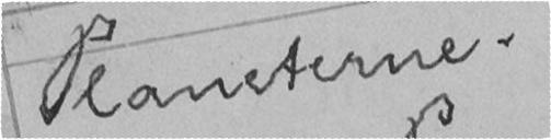Planeterne.
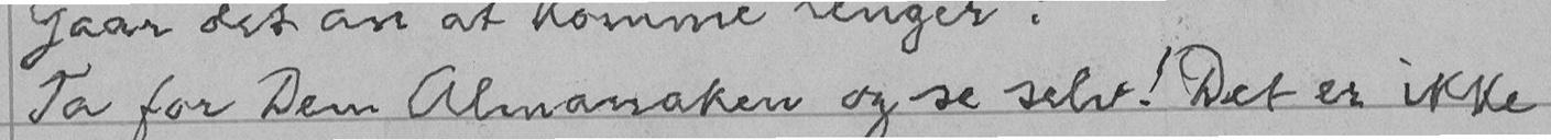Ta for Dem Almanaken og se selv! Det er ikke
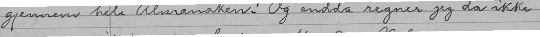gjennem hele Almanaken! Og endda regner jeg da ikke
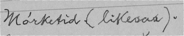Mørketid (likesaa).
